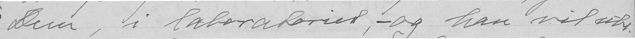Dem, i laboratoriet, - og han vil ube-
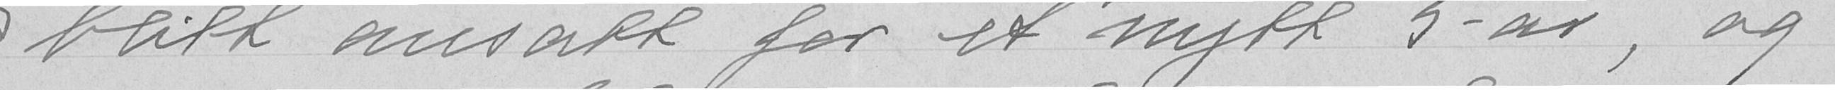blitt ansatt for et nytt 5-år, og
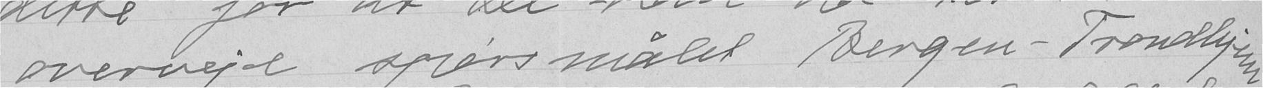overveje spørsmålet Bergen-Trondhjem
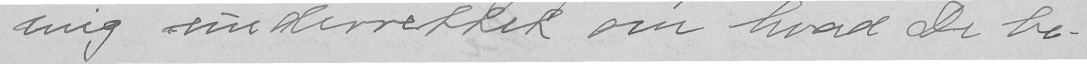mig underrettet om hvad De be-
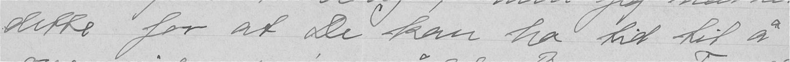dette for at De kan ha til til å
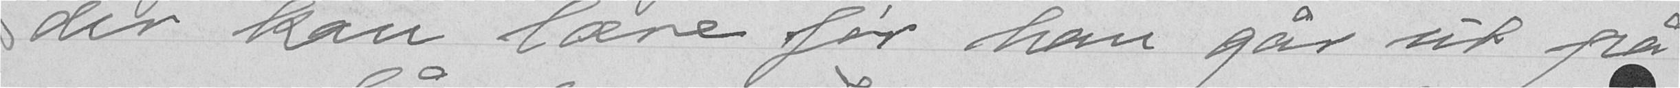der kan lære før han går ut på
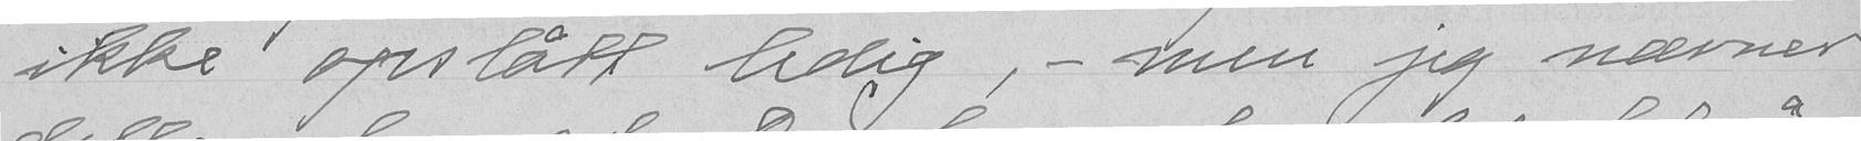ikke opslått ledig, - men jeg nævner
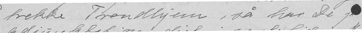trekke Trondhjem, så har De jo
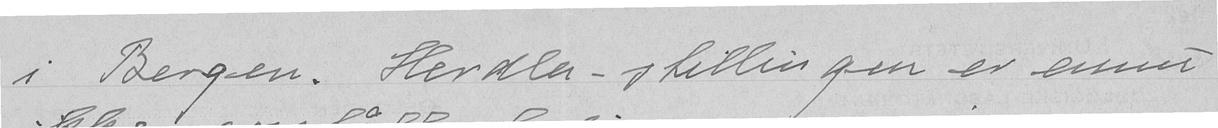i Bergen. Herdla-stillingen er ennu
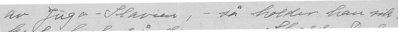av Jugo-Slavien, - så holder han sik-
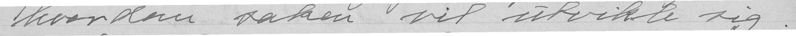hvordan saken vil utvikle sig.
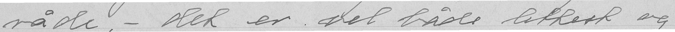råde, - det er dvl både lettestk og
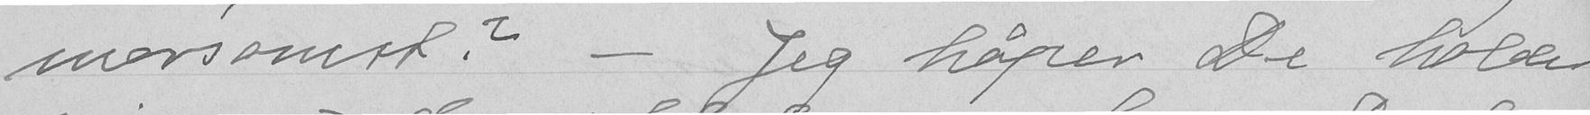morsomst? Jeg håper De holder
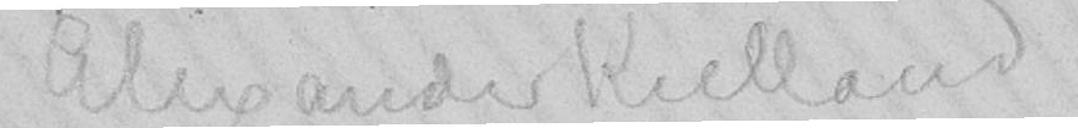Alexander Kielland
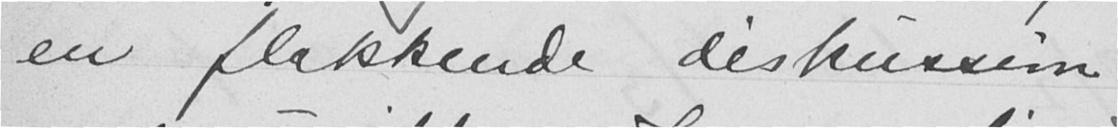en flakkende diskussion
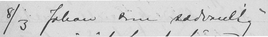8/3 Johan som sædvanlig
